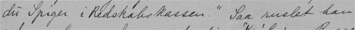du Spiger i Redskabskassen. Saa ruslet han
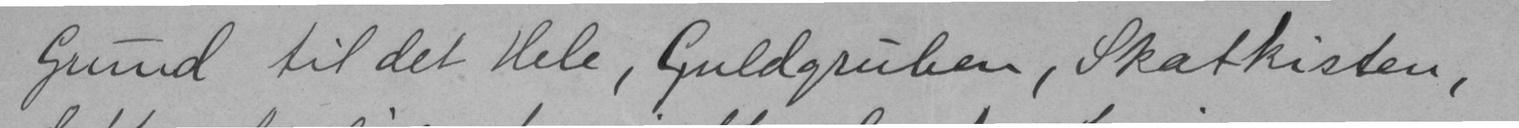Grund til det Hele, Guldgruben, Skatkisten,
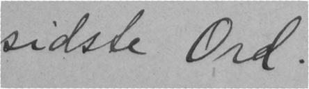sidste Ord.
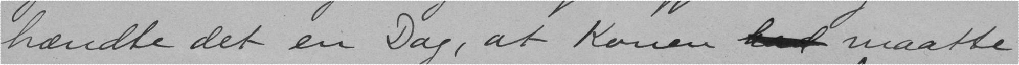hændte det en Dag, at Konen had maatte
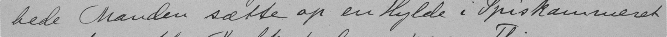bede Manden sætte op en Hylde i Spiskammeret
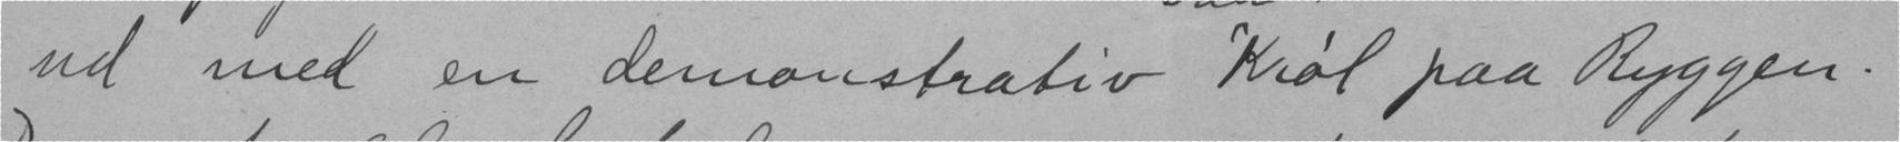ud med en demonstrativ Krøl paa Ryggen.
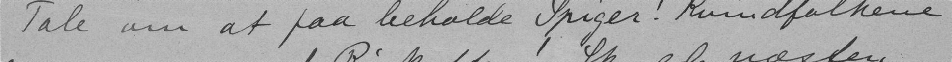Tale om at faa beholde Spiger! Kvindfolkene
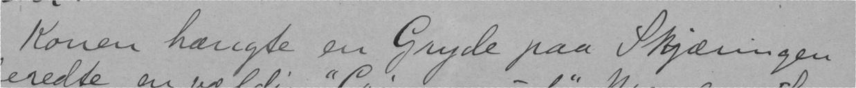Konen hængte en Gryde paa Skjæringen
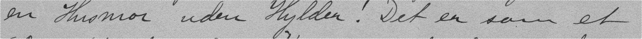en Husmor uden Hylder! Det er som et
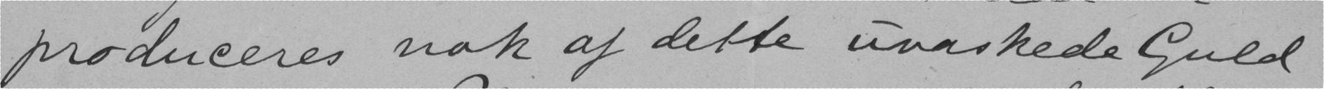produceres nok af dette uvaskede Guld
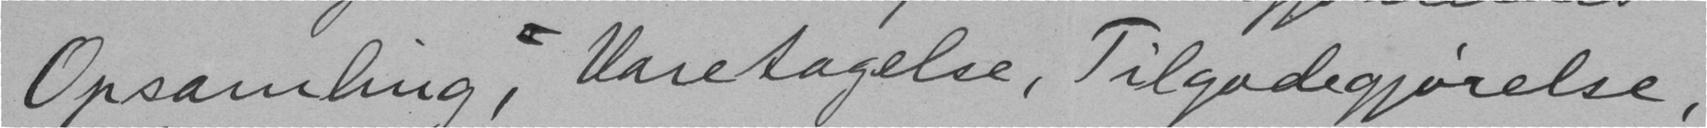Opsamling, Varetagelse, Tilgodegjørelse,
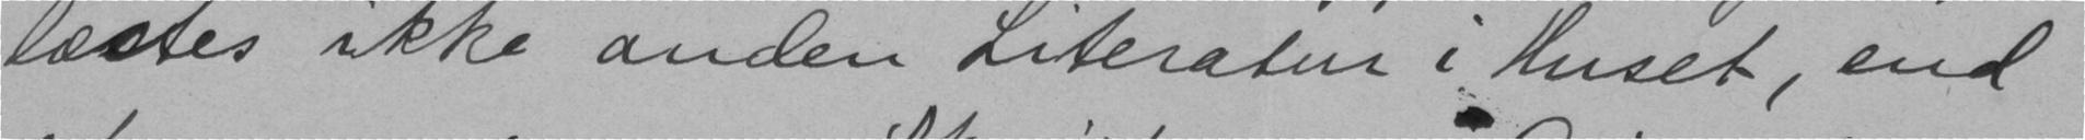læstes ikke anden Literatur i Huset, end
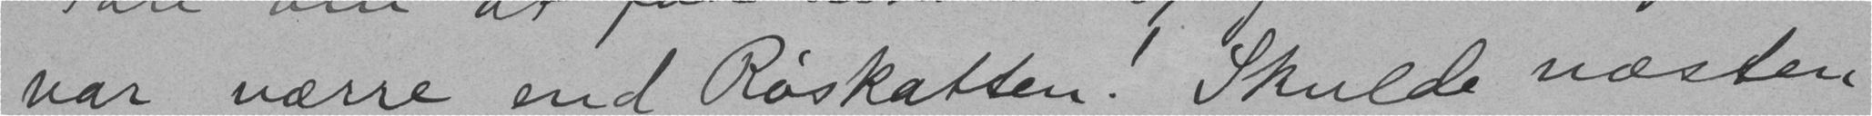har værre end Røskatten! Skulde næsten
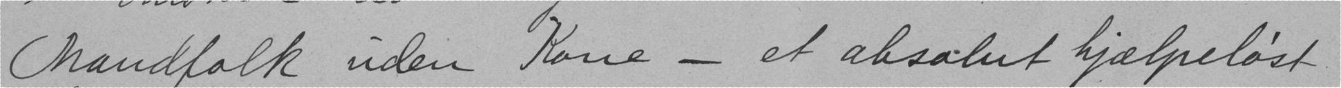Mandfolk uden Kone - et absolut hjælpeløst
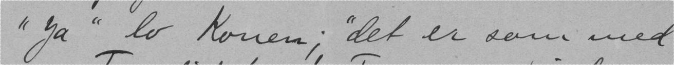"Ja" lo Konen; det er som med
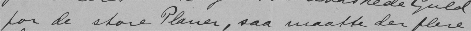for de store Planer, saa maatte der flere
